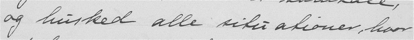og husked alle situationer, hvor
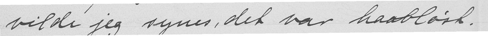vilde jeg synes, det var haabløst.
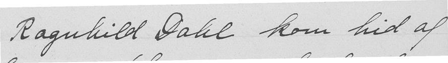Ragnhild Dahl kom hid af
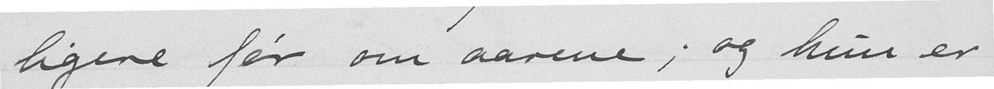ligere før om aarene; og hun er
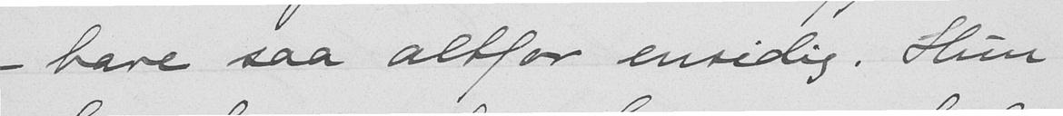- bare saa altfor ensidig. Hun
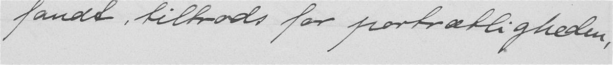fandt, tiltrods for portrætligheden,
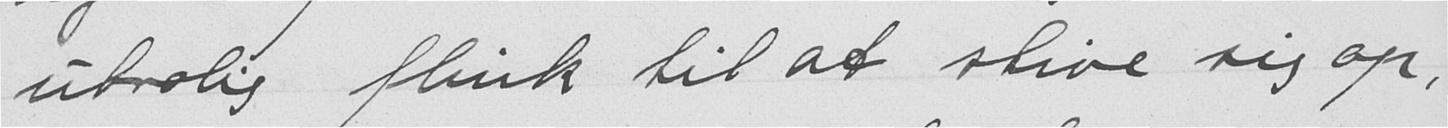utrolig flink til at stive sig op,
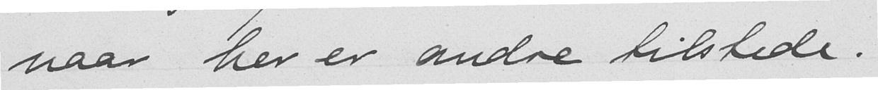naar her er andre tilstede.
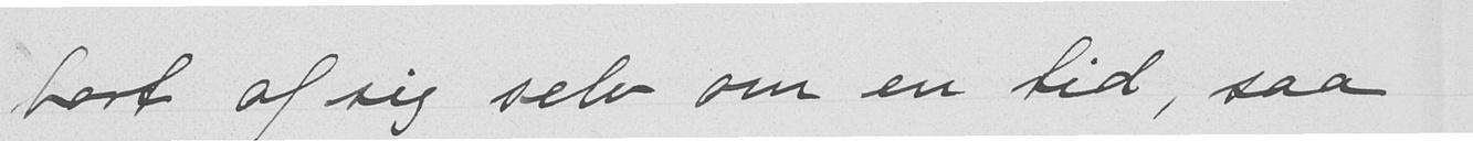bort af sig selv om en tid, saa
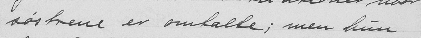søstrene er omtalte; men hun
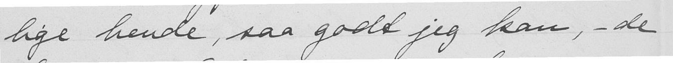lige hende, saa godt jeg kan, - de
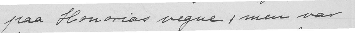paa Honorias vegne, men var
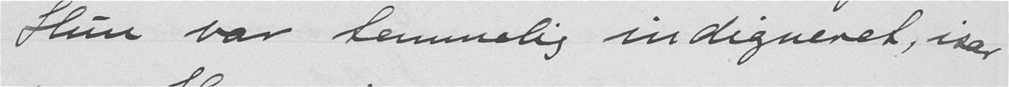Hun var temmelig indigneret, især
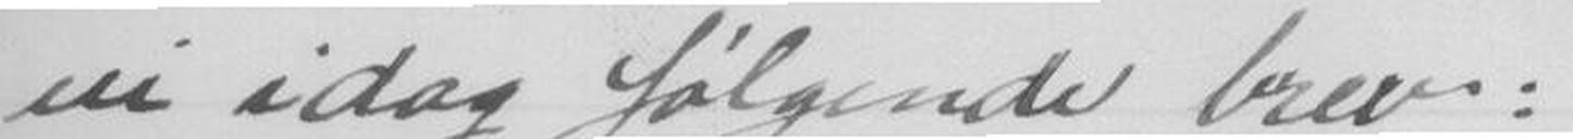vi idag følgende brev:
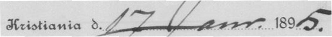Kristiania d. 17 Janr. 1895.
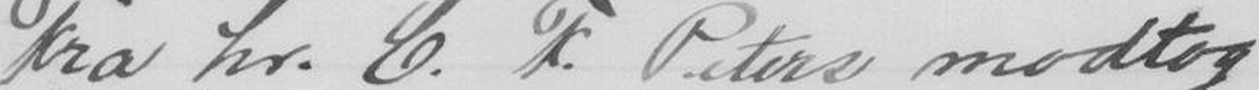Fra hr. C. F. Peters modtog
